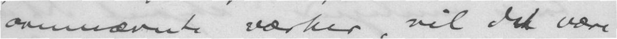ovennæmte værker, vil det være
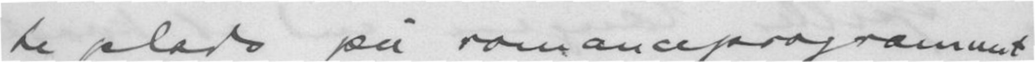te plads på romanceprogrammet
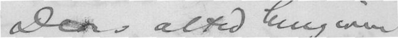Deres altid hengivne
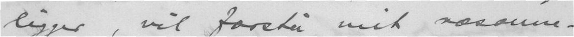ligger, vil forstå mit ræsonne-
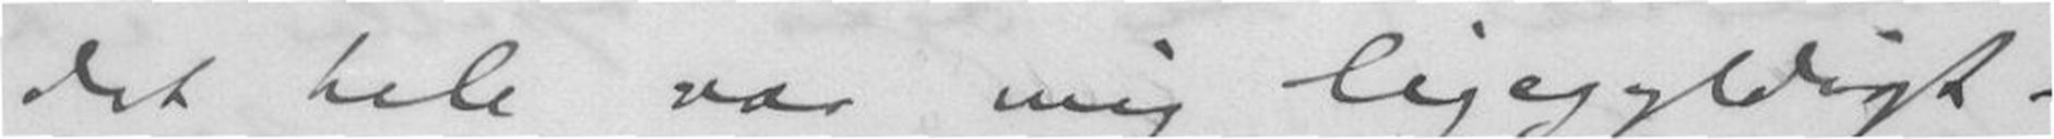det hele var mig ligegyldigt -
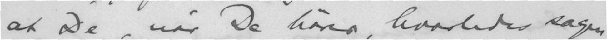at De, når De hører, hvorledes sagen
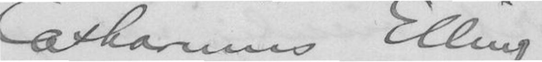Catharinus Elling
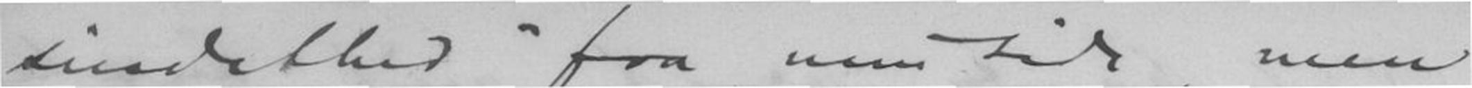sindethed" fra min side, men
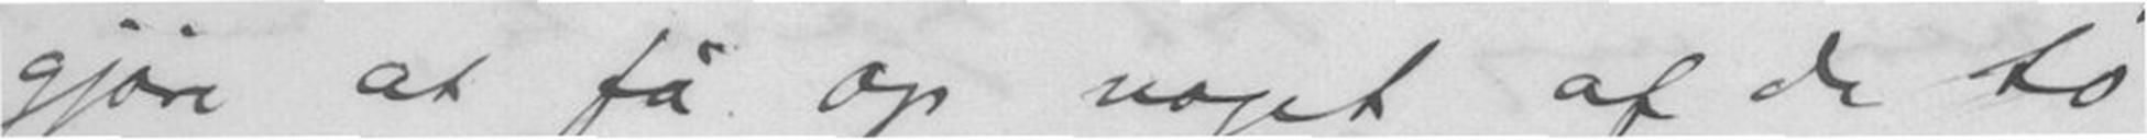gjøre at få op noget af de to
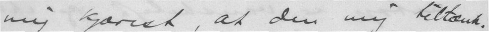mig kjærest, at den mig tiltænk-
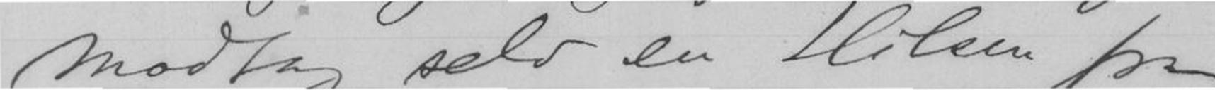modtag selv en Hilsen fra
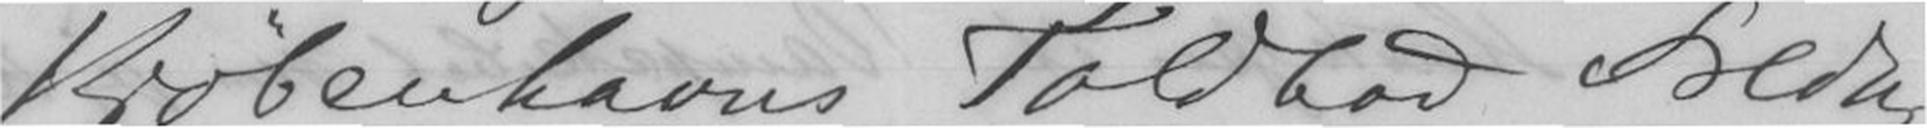Kjøbenhavns Tolbod Fredag
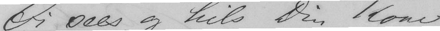vi sees og hils Din Kone
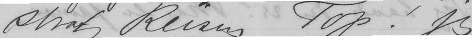stort Reisens Top! Jeg
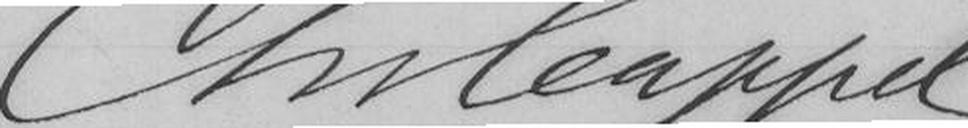Chr. Cappelen
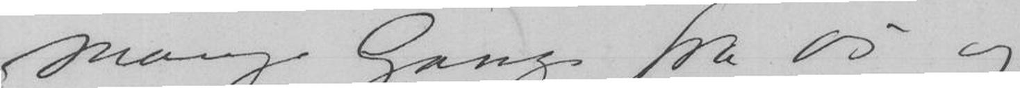mange Gange fra os og
